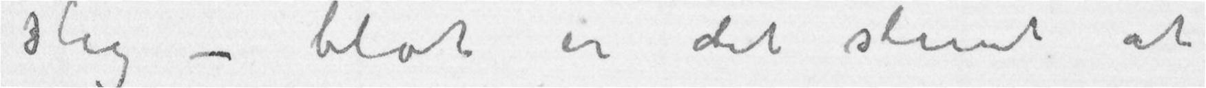slig – blot er det slemt at
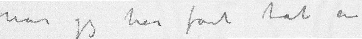når jeg har fået tat en
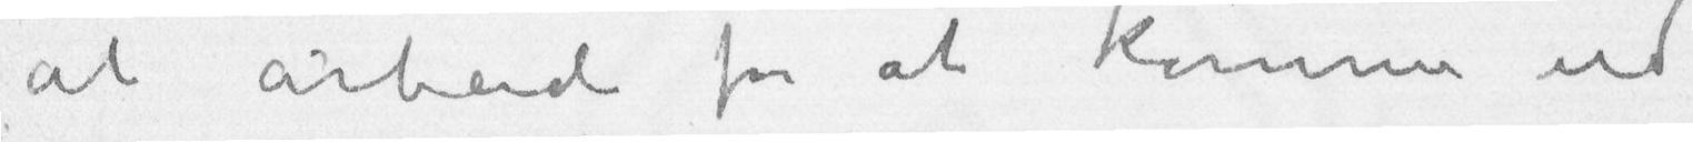at arbeide for at komme ud
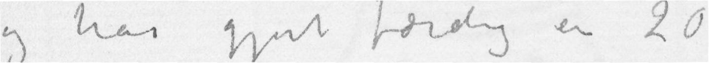og har gjort færdig en 20
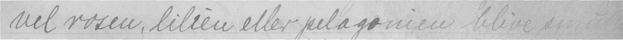vel rosen, lilien eller pelagonien blive smukest
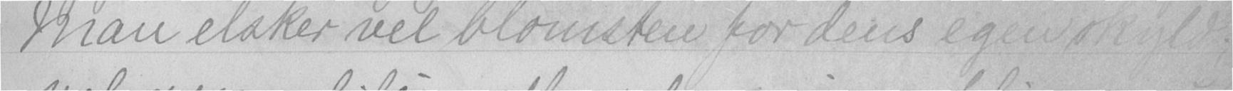Man elsker vel blomsten for dens egen skyld;
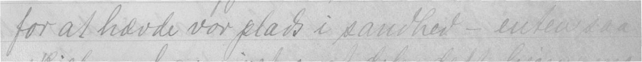for at hævde vor plads i sandhed - enten saa
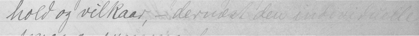hold og vilkaar, - dernæst den individuelle
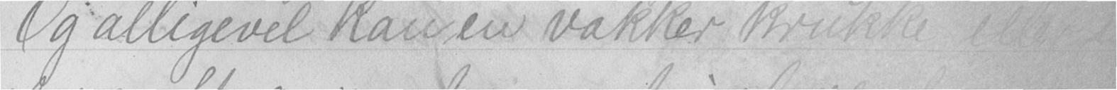Og alligevel kan en vakker krukke ellers
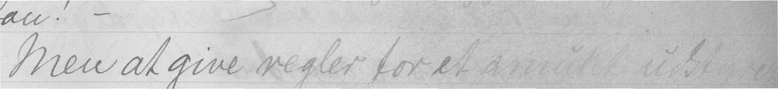Men at give regler for et smukt udstyret
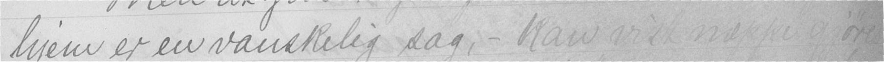hjem er en vanskelig sag, - kan vist næppe gjøre
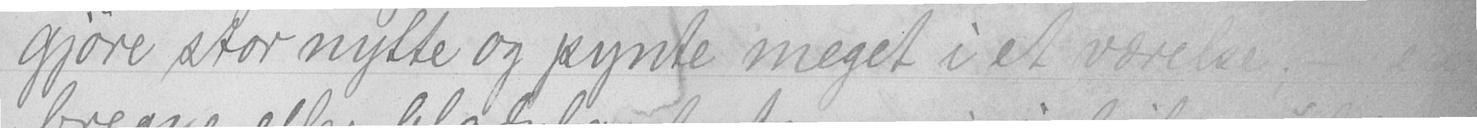gjøre stor nytte og pynte meget i et værelse; - en
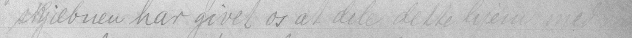skjæbnen har givet os at dele dette hjem med en
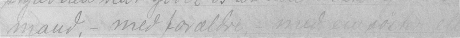mand, - med forældre, - med en søster eller med
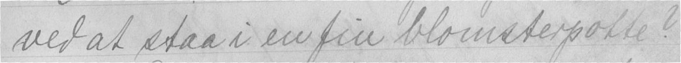ved at staa i en fin blomsterpotte?
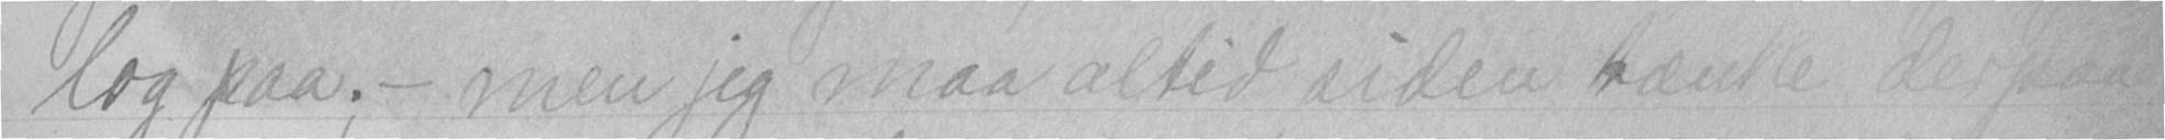log paa; - men jeg maa altid siden tænke derpaa
